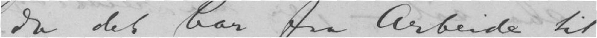da det bar fra Arbeide til
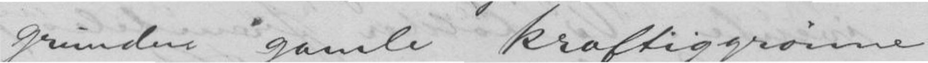grunden gamle kraftiggrønne
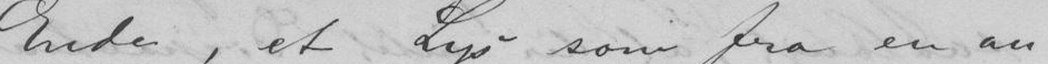Ende, et Lys som fra en an-
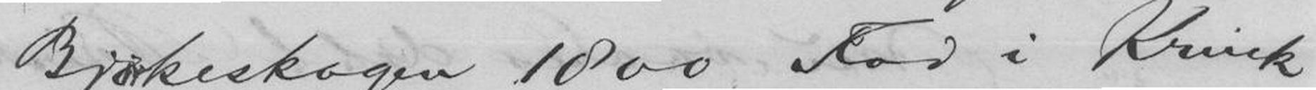Bjørkeskogen 1000 Fod i Krink
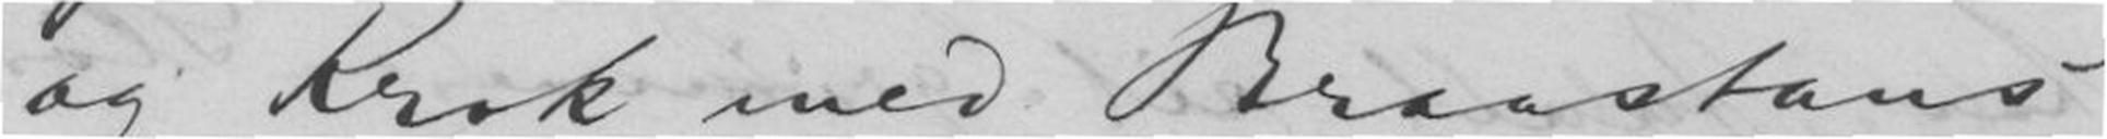og Krok med Braastans
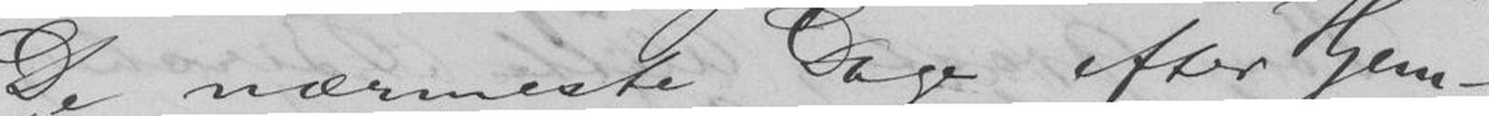De nærmeste Dage efter Hjem-
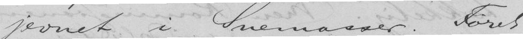jevnet i Snemasser. Føret
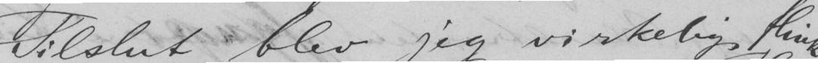Tilslut blev jeg virkelig flink.
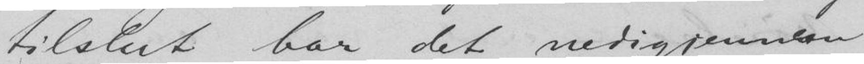tilslut bar det nedigjennem
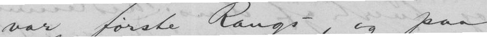var første Rangs, og paa
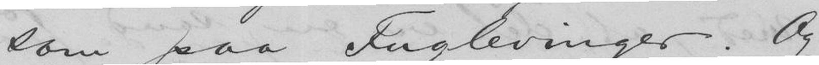som paa Fuglevinger. Og
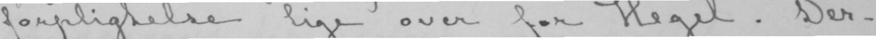forpligtelse lige over for Hegel. Der-
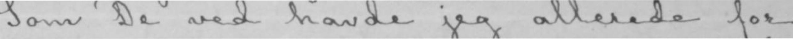Som De ved havde jeg allerede for
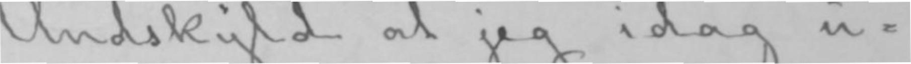Undskyld at jeg idag u-
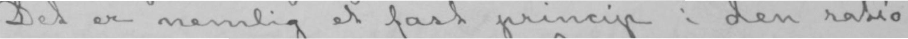Det er nemlig et fast princip i den ratio-
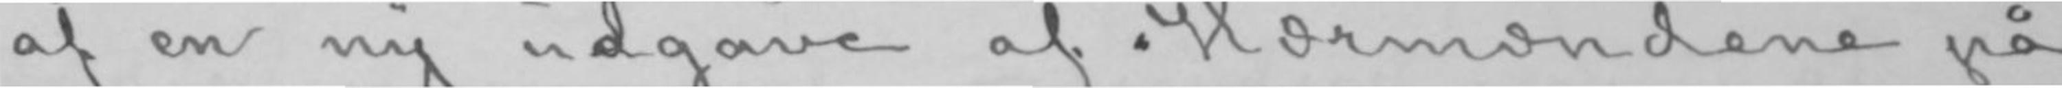af en ny udgave af «Hærmændene på
